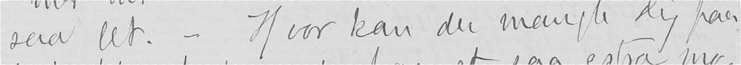saa let. - Hvor kan der mangle Dig paa
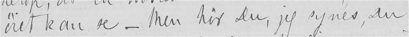øiet kan se. - Men hør Du, jeg synes, Du
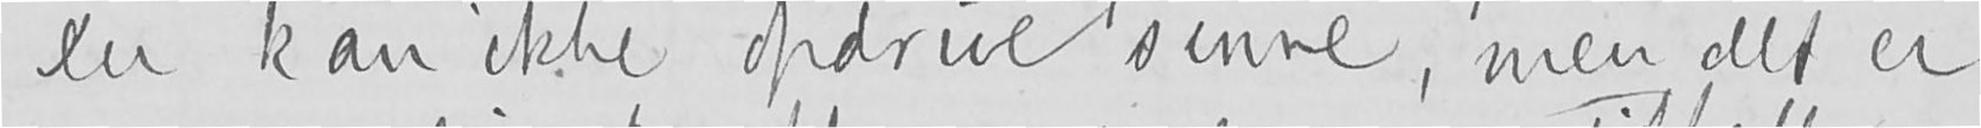Du kan ikke opdrive sinne, men det er
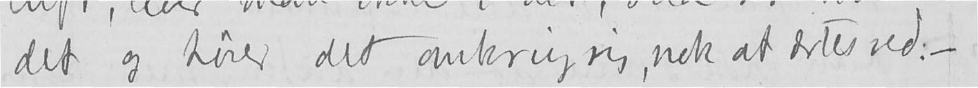det og hører det omkring sig, nok at ærtes ved. -
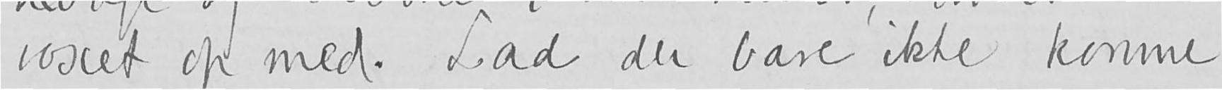voxet op med. Lad der bare ikke komme
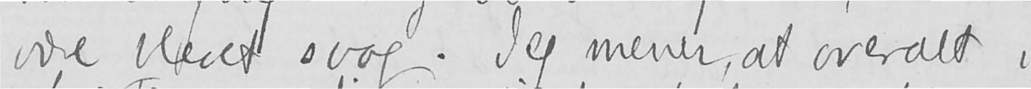være blevet svag. Jeg mener, at overalt i
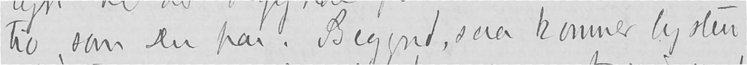tiv, som Du har. Begynd, saa kommer lysten
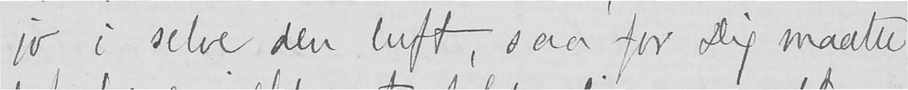jo i selve den luft, saa for Dig maatte
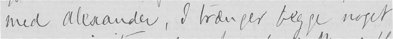med Alexander, I trænger begge noget
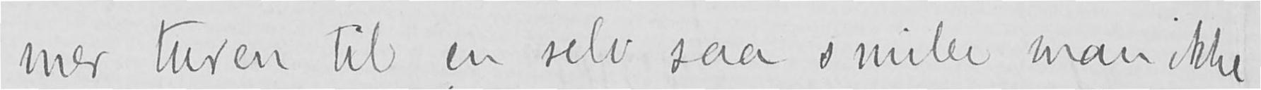mer turen til en selv saa smiler man ikke
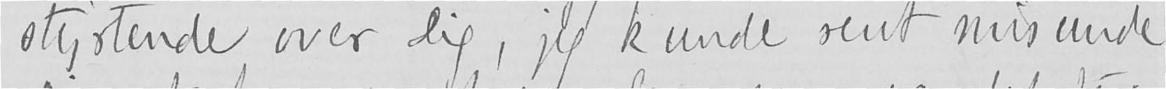styrtende over dig, jeg kunde rent misunde
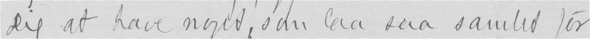dig at have noget, som laa saa samlet for
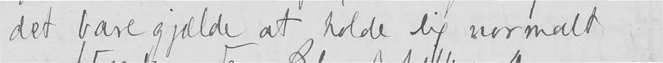det bare gjælde at holde dig normalt
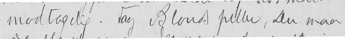modtagelig. Tag Blonds piller, Du maa
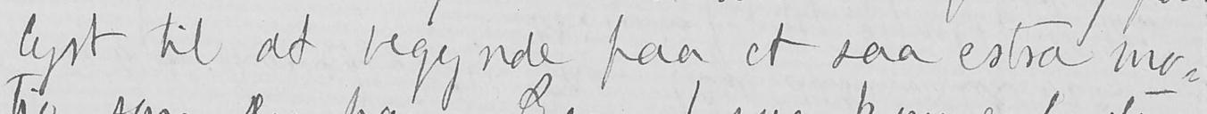lyst til at begynde paa et saa extra mo-
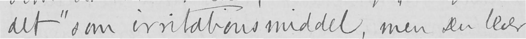det" som irritationsmiddel, men Du lever
